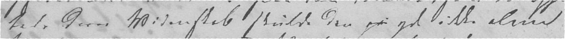tede deres Videnskab skulde den yde ikke alene
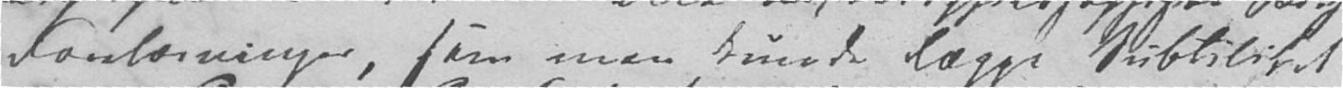Forelæsninger, som man kunde lægge Subtilitet
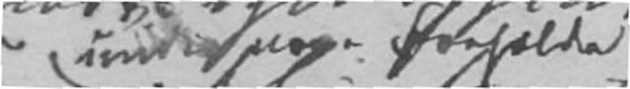under vore Forholde
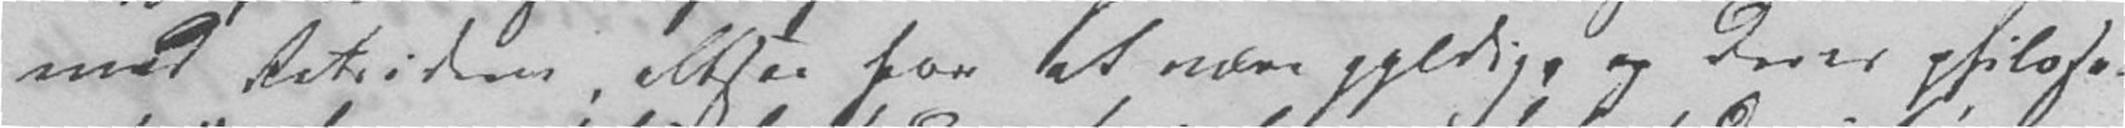med Retsideen, altsaa for at være gyldig. Deres philoso-
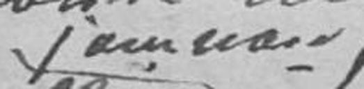som vare
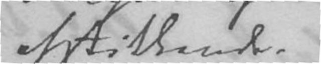afstikkende.
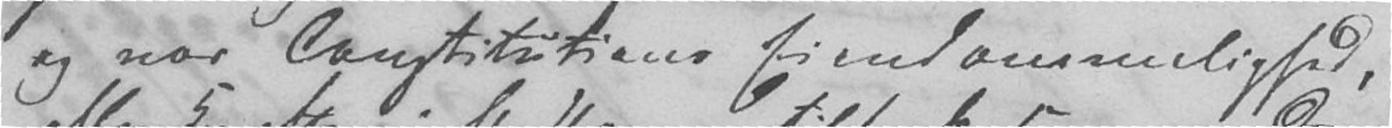og vor Constitutions Eiendommelighed.
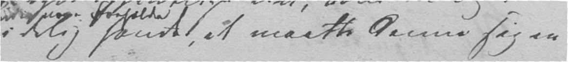idelig hændte, at maatte danne sig en
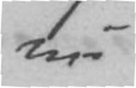nu
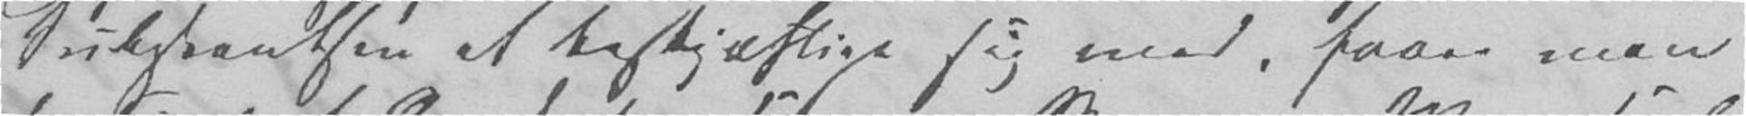Substantsen at Beskjæftige sig med, faar man
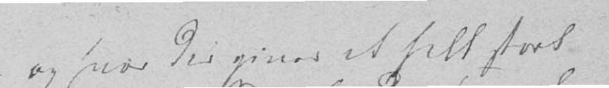og hvor der gives et helt stort
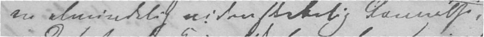en almindelig videnskabelig Dannelse,
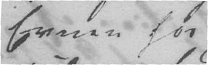Evnen hos
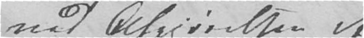ved Afgjørelsen af
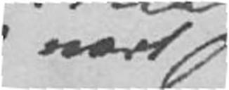vort
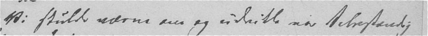Vi skulde værne om og udvikle vor Selvstændig-
